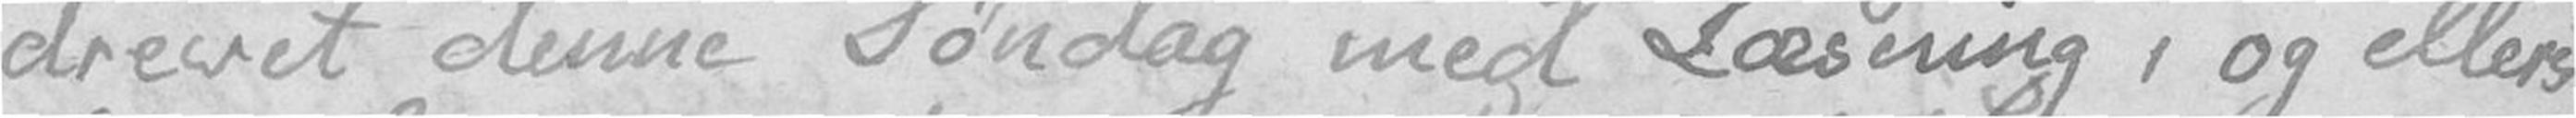drevet denne Søndag med Læsning, og ellers
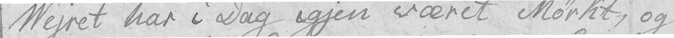Vejret har i Dag igjen været Mørkt, og
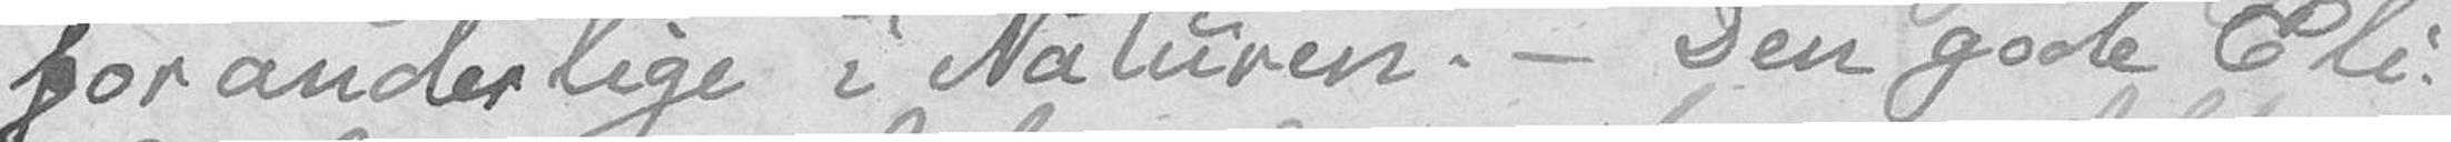foranderlige i Naturen. – Den gode Eli
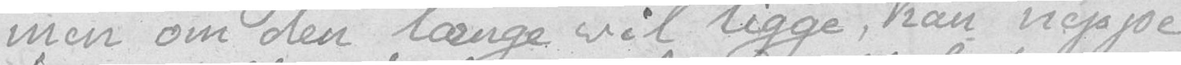men om den længe vil ligge, kan neppe
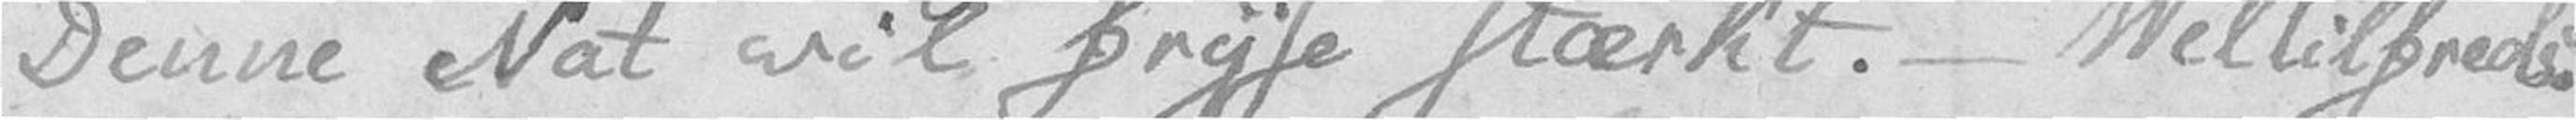Denne Nat vil fryse stærkt. – Veltilfreds.
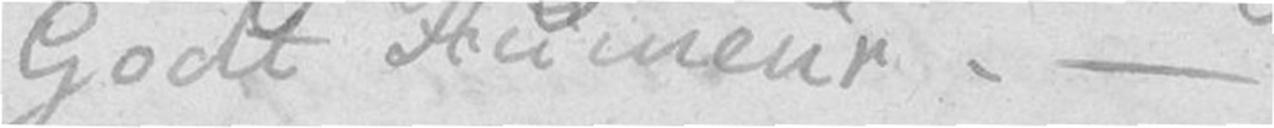Godt Humeur. –
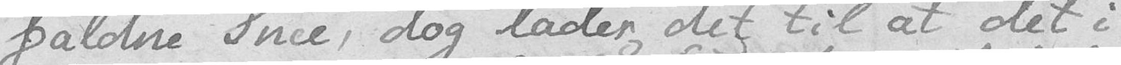faldne Snee, dog lader det til at det i
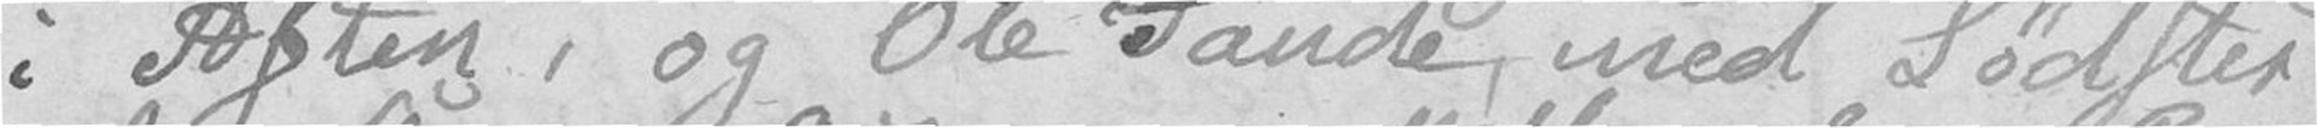i Aften, og Ole Tande med Sødster
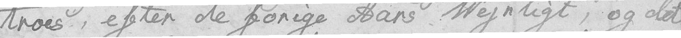troes, efter de forige Aars Vejrligt, og det
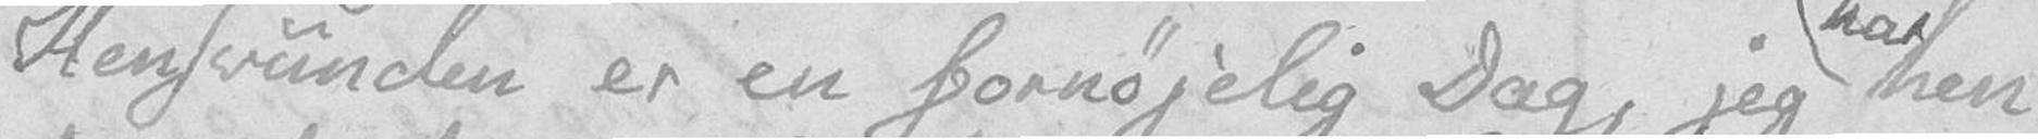Hensvunden er en fornøjelig Dag, jeg har hen
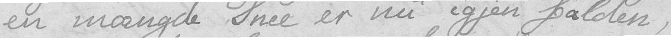en mængde Snee er nu igjen falden,
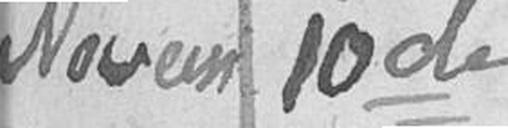Novem 10de
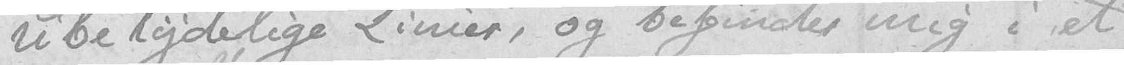ubetydelige Linier, og befinder mig i et
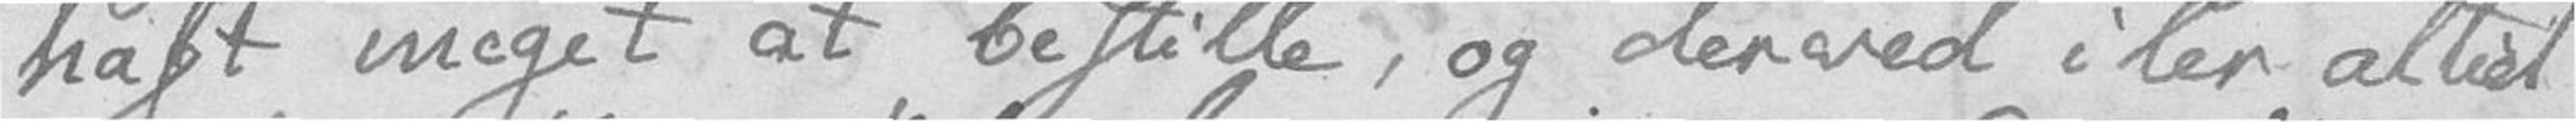haft meget at bestille, og derved iler altid
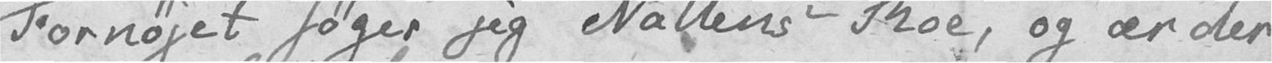Fornøjet søger jeg Nattens Roe, og er der
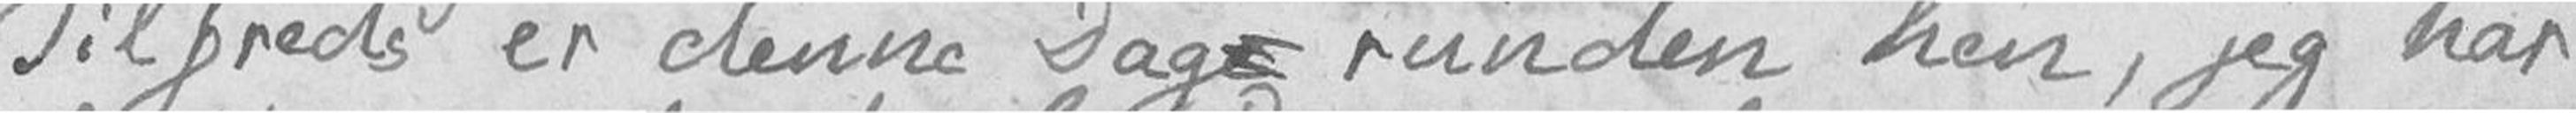Tilfreds er denne Dag runden hen, jeg har
